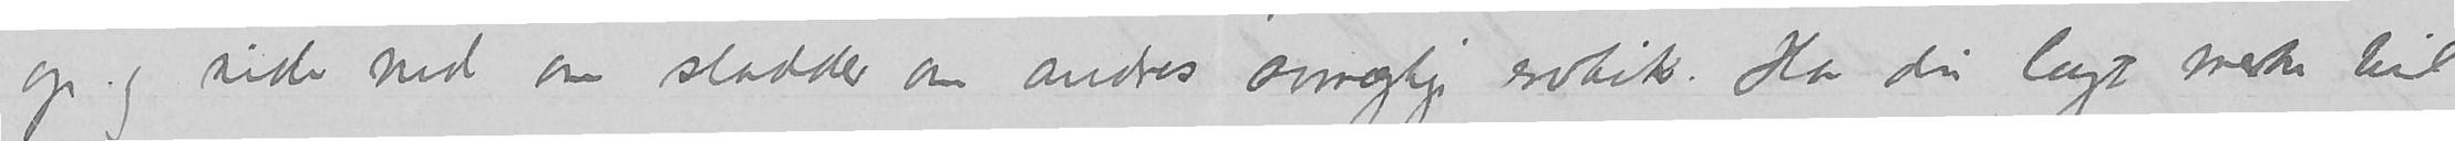op og side ned om sladder om andres avmægtige erotik. Har du lagt merke til
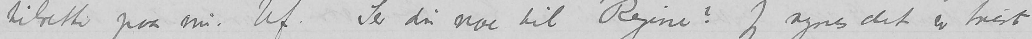tilrette paa nu. Uf.  Ser du noe til Regine? Jeg synes det er trist at se,
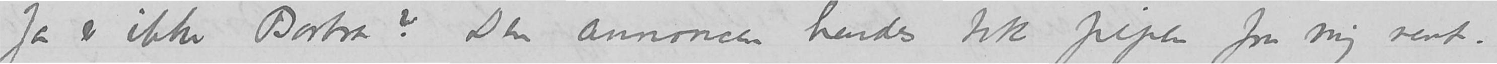Ja er ikke Barbra? Den annoncsen hendes tok pipen fra mig rent.
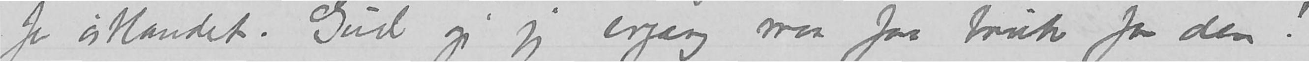fra utlandet.  Gud gi jeg engang maa faa bruk for den!
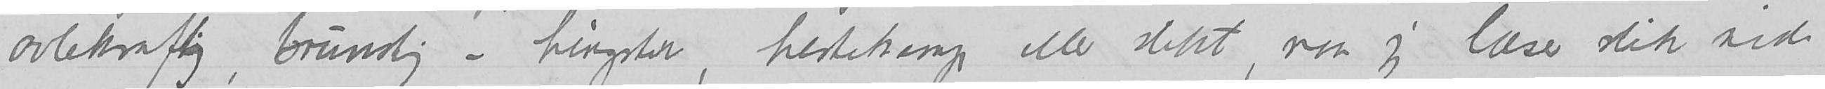avlekraftig, brunstig –- hingster, hestekamp eller slikt, naar jeg læser slik side op og side ned <om> sladder om andres sørgelige avmægtige erotik.  Har du lagt merke til at der er aldrig nogen som bedriver nogenting i Kincks bøker,
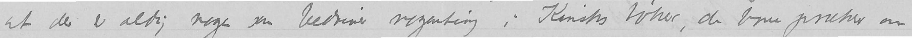at der er aldrig nogen som bedriver nogenting i Kincks bøker, de bare prækater om
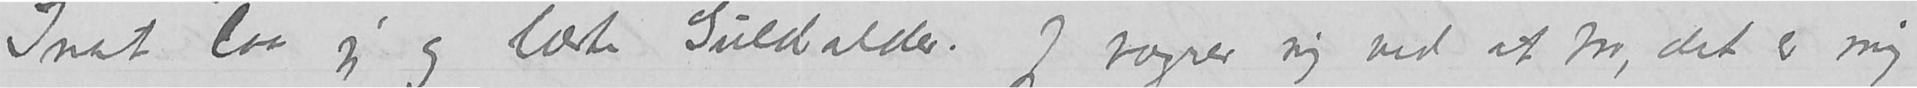Inat laa jeg og læste Guldalder.  Jeg vægrer mig ved at tro, det er mig
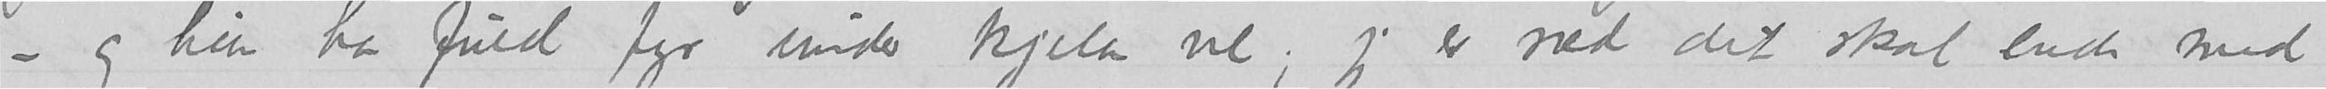og hun har fuld fyr under kjelen vel; jeg er ræd det skal ende med
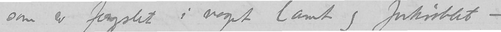som er fengslet i noget tamt og forkrøblet -
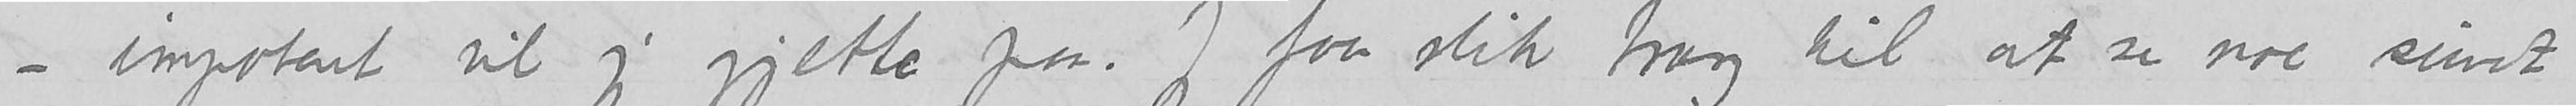impotent vil jeg gjette paa. Jeg faar slik trang til at sei noe sundt
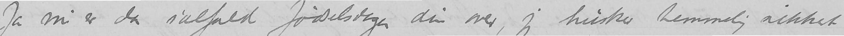Ja nu er da ialfald fødselsdagen din over, jeg husker temmelig sikkert
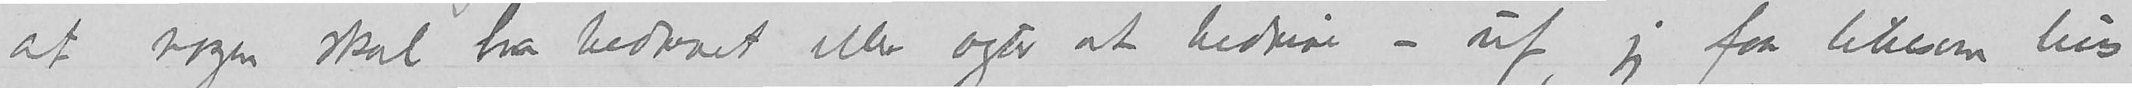at nogen skal ha bedrevet eller agter at bedrive - uf, jeg faar likesom lus
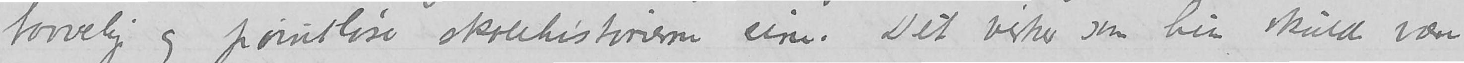tarvelige og pøointløse skolehistorierne sine.  Det virker som hun skulde være
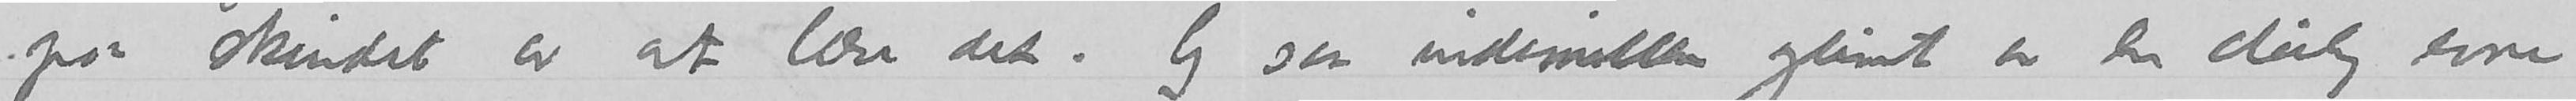på skindet av at læse det. Jeg ser indimellem glimt av en deilig evne
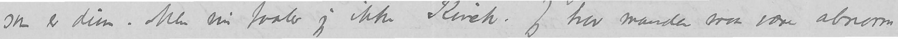som er dum. Men nu taaler jeg ikke Kinck.  Jeg tror manden maa være abnorm
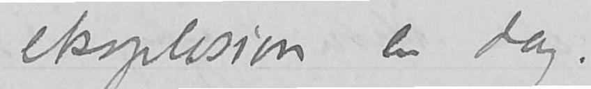eksplosion en dag.
